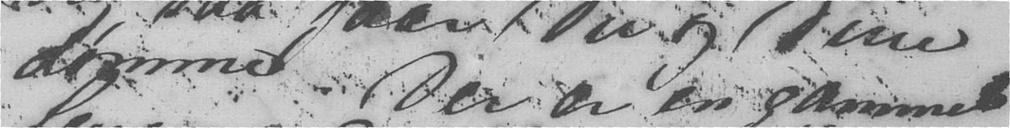dømme. Der er en gammel
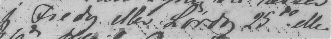jeg Fredag eller Lørdag 25de eller
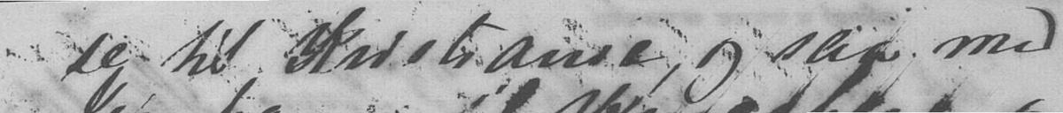se til Kristiania, og slig med
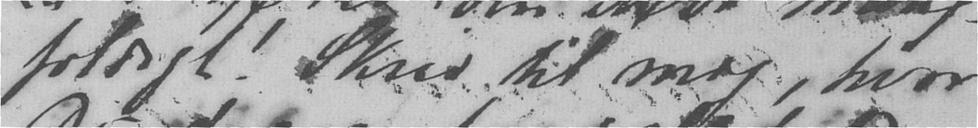foldigt! Skriv til mig, hvor
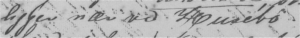ligger nær ved Husebø.
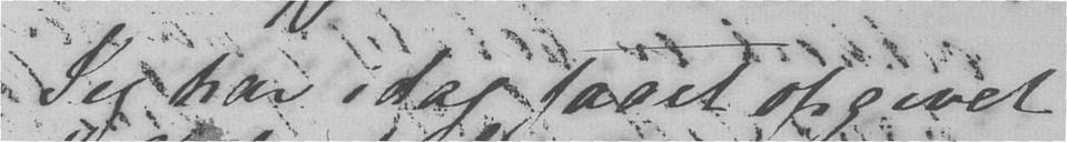Jeg har idag faaet opgivet
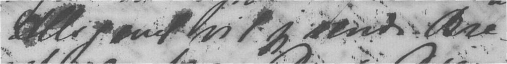Alligevel vil jeg sende Bre-
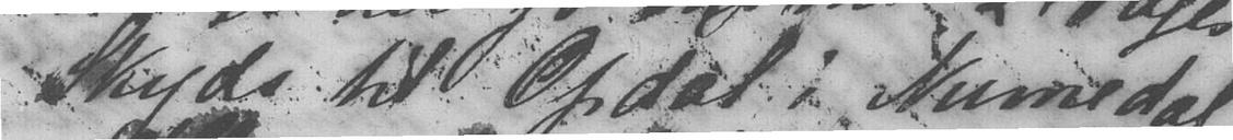Skyds til Opdal i Numedal.
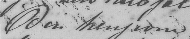Din hengivne
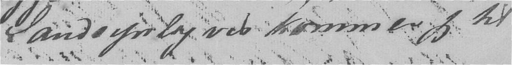Sandsynligvis kommer jeg til
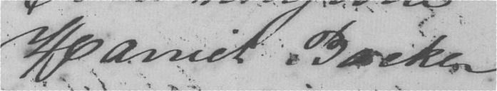Harriet Backer
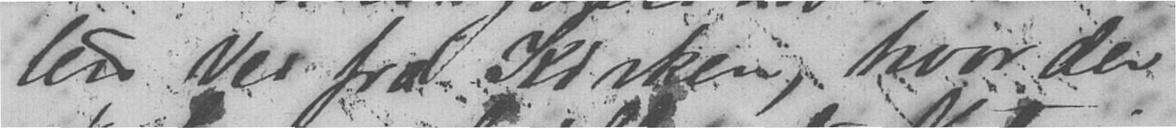ters Vei fra Kirken, hvor der
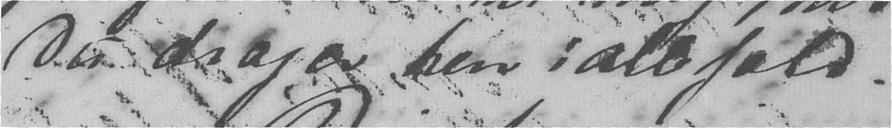Du drager hen iallefald.
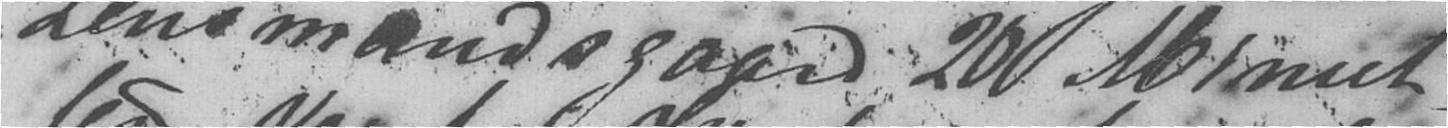Lensmandsgaard 20 Minut-
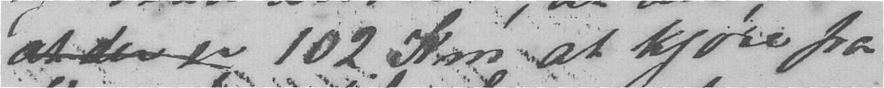at der er 102 Km at kjøre fra
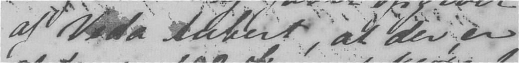af Veda Aubert, at der er
